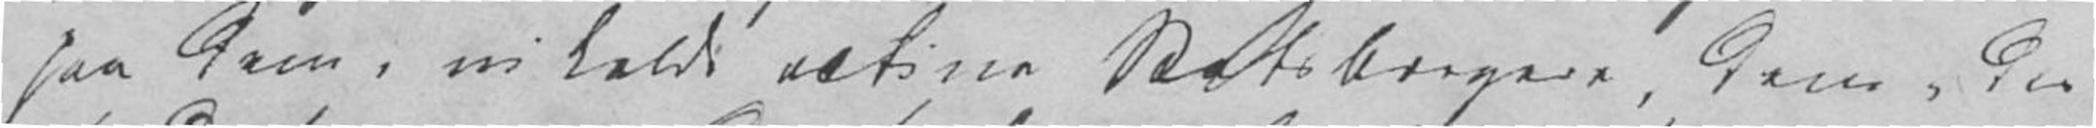paa dem, vi kalde active Statsborgere, dem, der
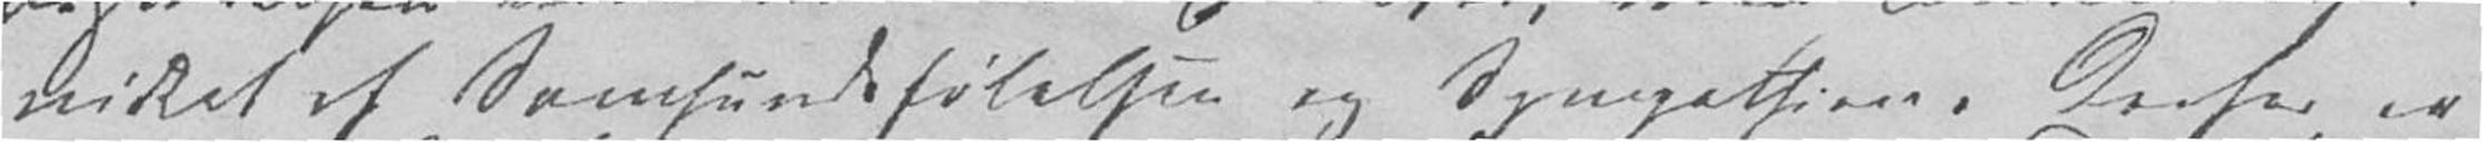nødet af Samfundsfølelsen og Sympathien. Derfor er
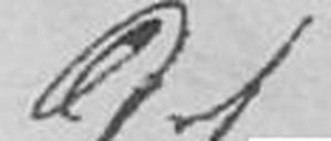det
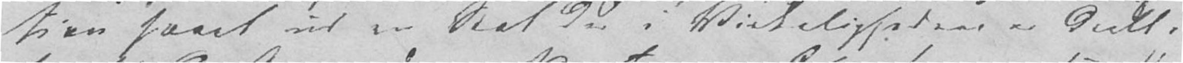tion faaet ud en Stat der i Virkeligheden er deelt i
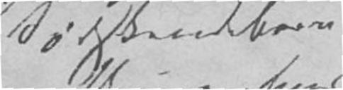Sødskendbarn,
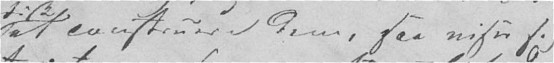at construere dem, saa viser sig
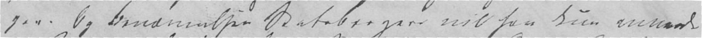ger. Og Benævelsen Statsborgere vil han kun anvende
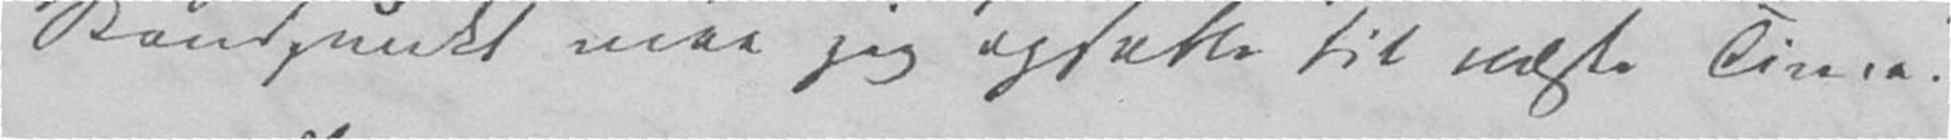Standpunkt maa jeg opsætte til næste Time.
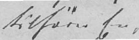tilhøre En,
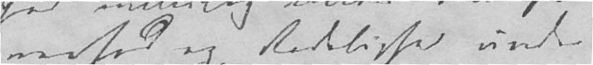renhed og Redelighed under
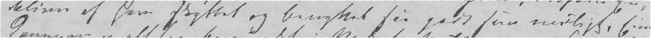bliver af ham skjøttet og benyttet saa godt som muligt, Eien-
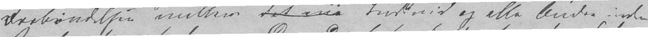Forbindelsen mellem det ene Individ og alle Andre inden
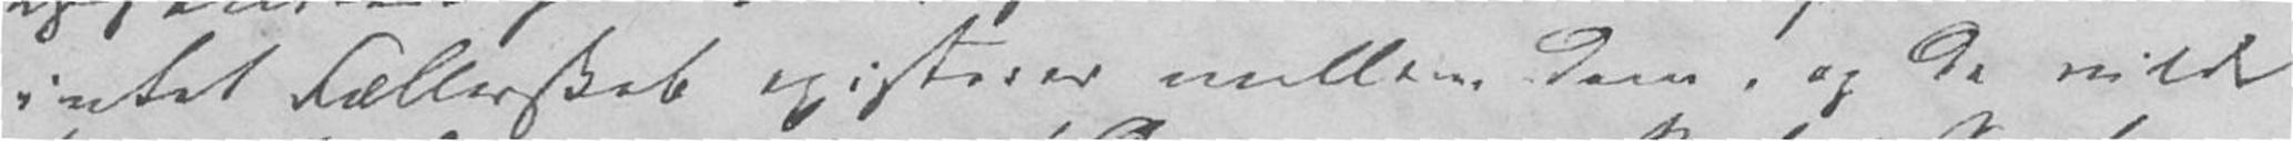intet Fællesskab existerer mellem dem, og de vilde
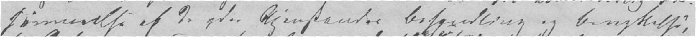sømmelse af de ydre Gjenstandes Behandling og Benyttelse,
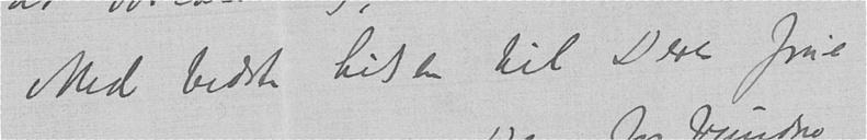Med bedste hilsen til Deres frue
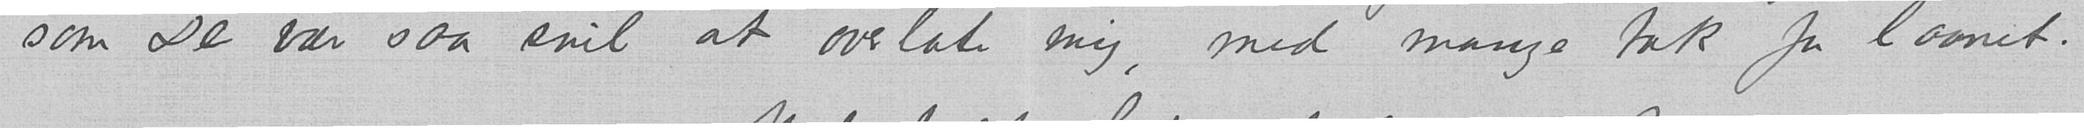som De var saa snil at overlate mig, med mange tak for laanet.
